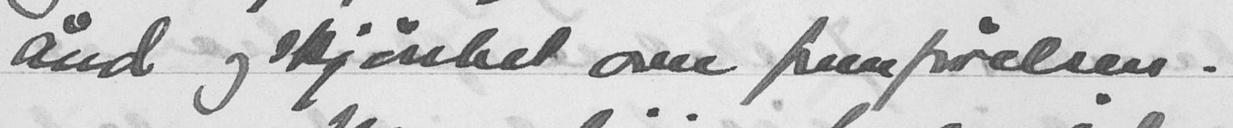ånd og skjønnhet over fremførelsen.
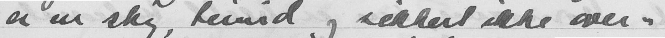er en sky, timid og sikkert ikke over-
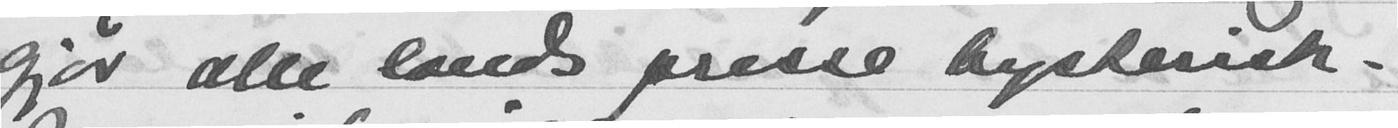gjør alle lands presse hysterisk.
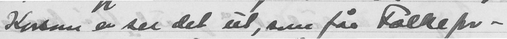Korsom er ser det ut, som får Folkefor-
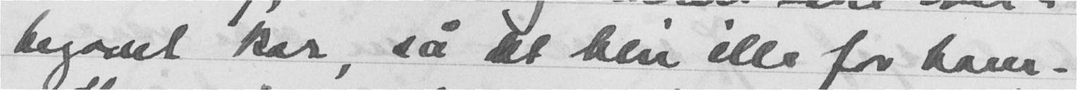begavet kar, så det blir ille for ham.
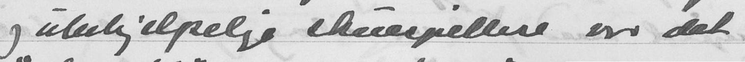og ubehjelpelige skuespillere var det
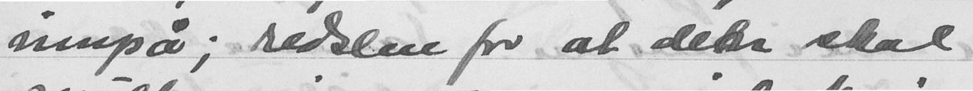innpå; redslen for at den skal
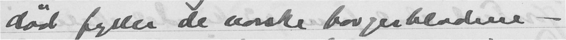død fyller de norske borgerbladene -
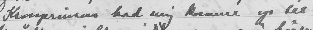Kronprinsen bad mig komme op til
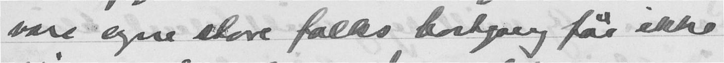våre egne store folks bortgang får ikke
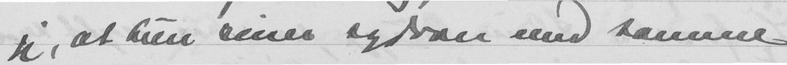jeg, at hun sener sygdvan med tomme
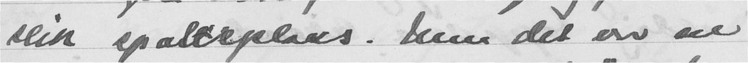slik spalteplass. Men det er en
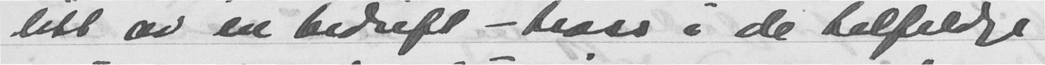litt av en bedrift - tross i de tilfeldige
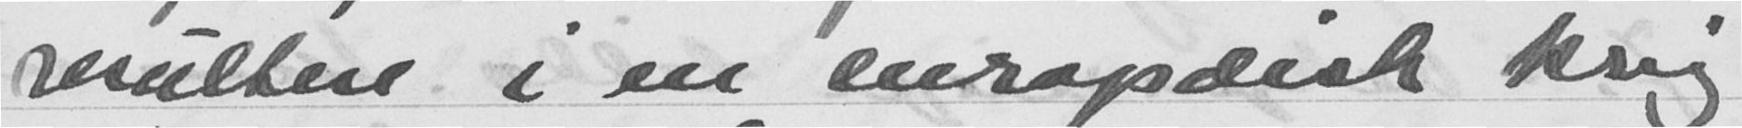resultere i en europeisk krig
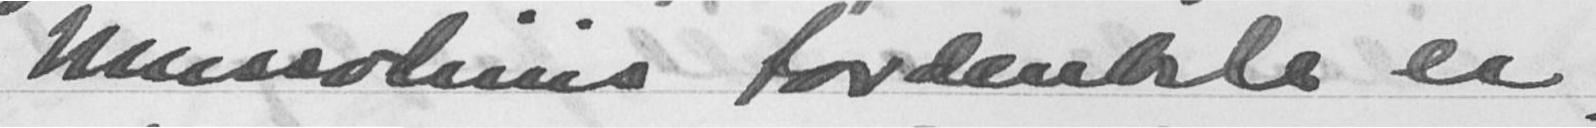Mussolinis tordentale er
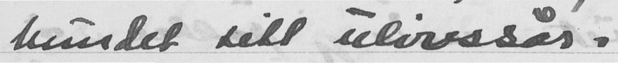bundet sitt ulivssår.
